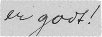er godt!
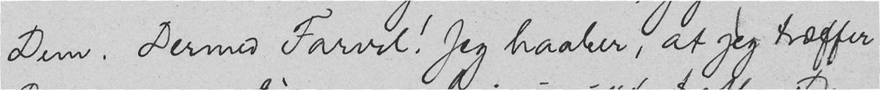Dem. Dermed Farvel! Jeg haaber, at jeg træffer
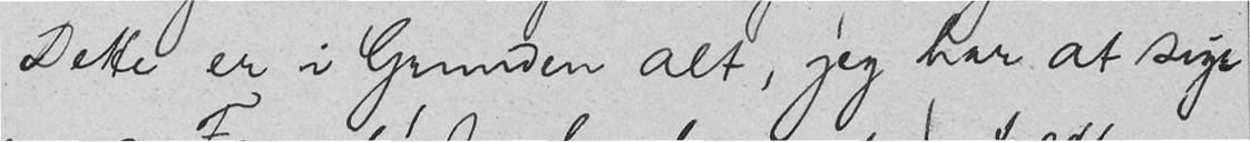Dette er i Grunden alt, jeg har at sige
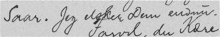Saar. Jeg elsker Dem endnu.
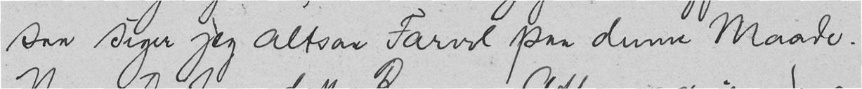saa siger jeg altsaa Farvel paa denne Maade.
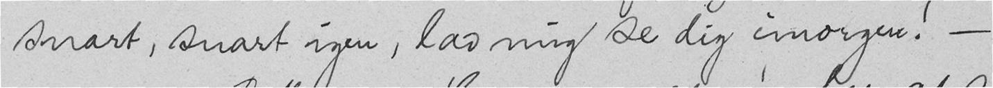snart, snart igen, lad mig se dig imorgen! -
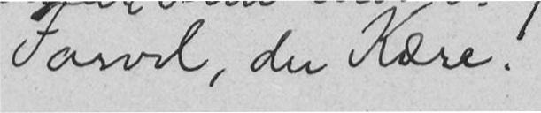Farvel, du Kære!
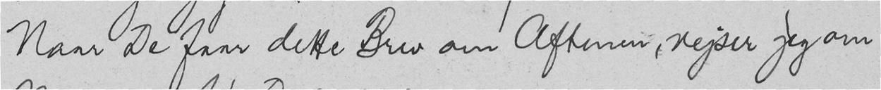Naar De faar dette Brev om Aftenen, rejser jeg om
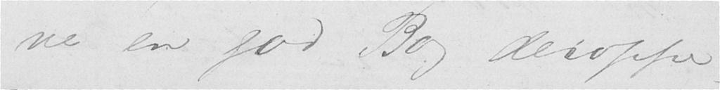ve en god Bog deroppe.
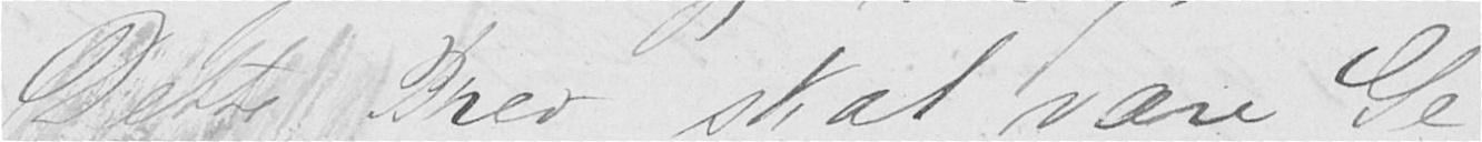Dette Brev skal være Ge-
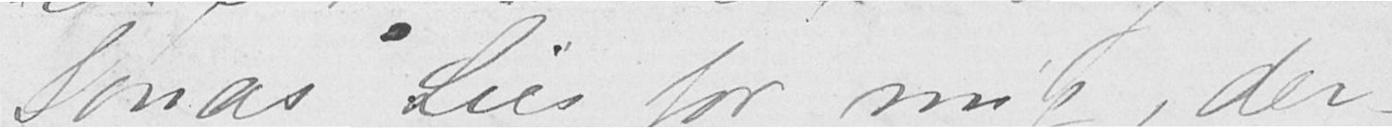Jonas Lies for mig, der-
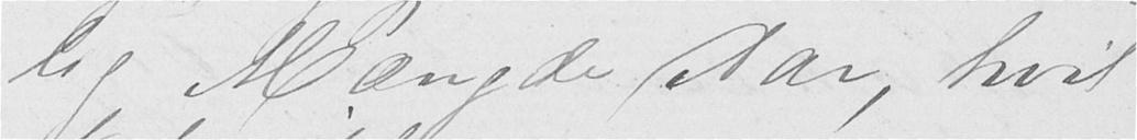lig Mængde Aar, hvil-
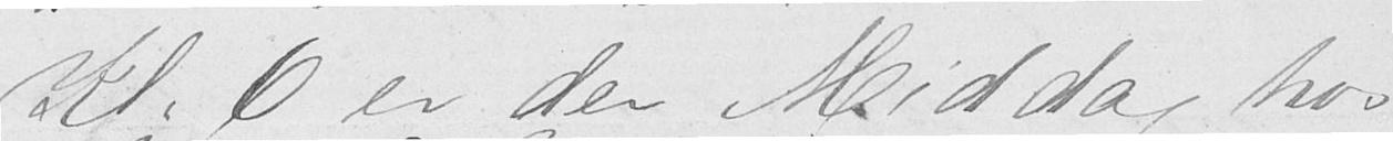Kl: 6 er der Middag hos
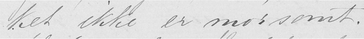ket ikke er morsomt.
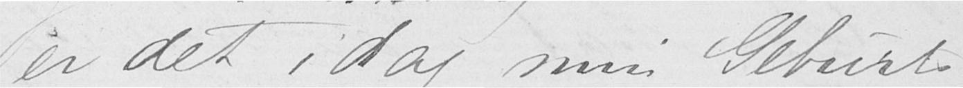er det i dag min Geburts-
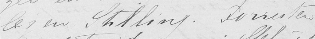legen Stilling. Forresten
